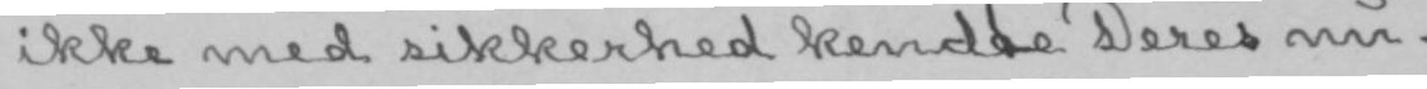ikke med sikkerhed kendete Deres nu-
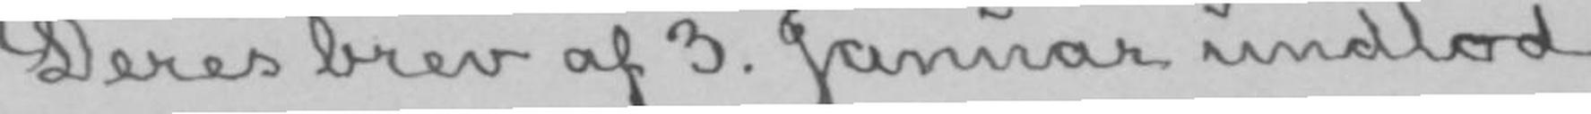Deres brev af 3. Januar undlod
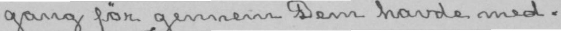gang før gennem Dem havde med-
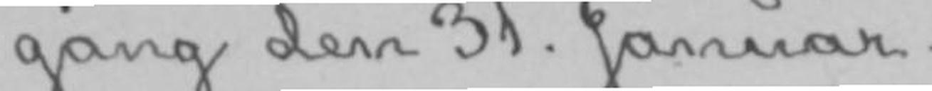gang den 31. Januar.
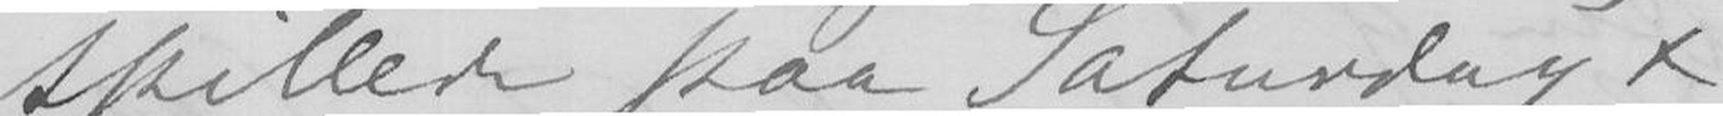spillede paa Saturday &
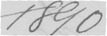1890
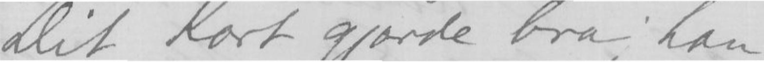Dit Kort gjorde bra; han
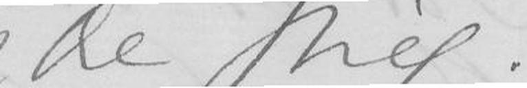Kjære Grieg.
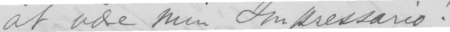at være min Impressario!
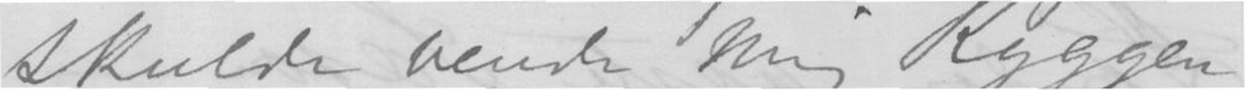skulde vende mig Ryggen,
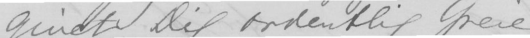givet Dig ordentlig Greie
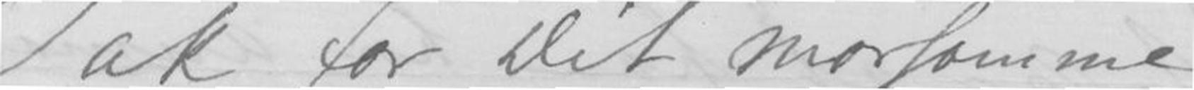Tak for Dit morsomme
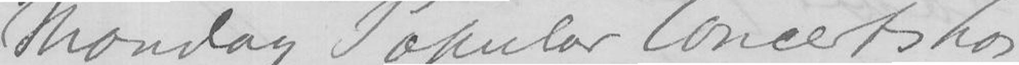Monday Popular Concert hos
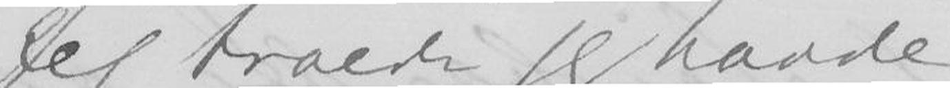Jeg troede jeg havde
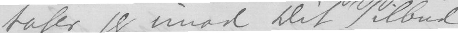tager jeg imod Dit Tilbud
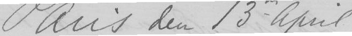Paris den 13de April
